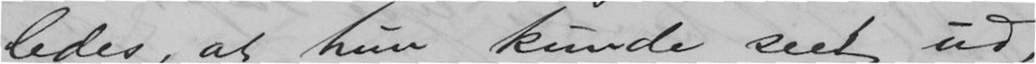ledes, at hun kunde seet ud,
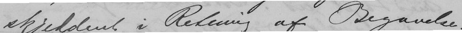skjeldent i Retning af Begavelse.
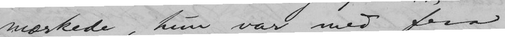mærkede, hun var med fra
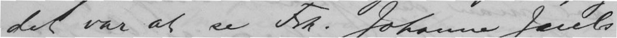det var at se Frk. Johanne Juels
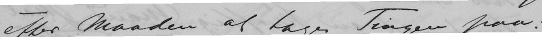efter Maaden at tage Tingen paa!
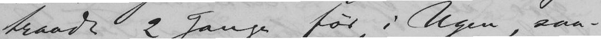traadt 2 Gange før i Ugen, saa-
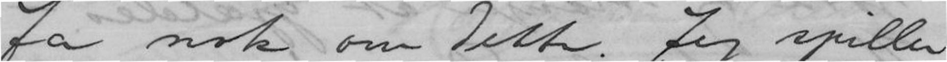Ja nok om dette. Jeg spiller
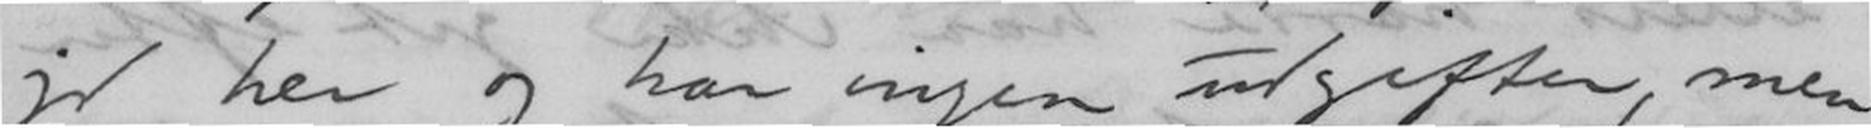jo her og har ingen udgifter, men
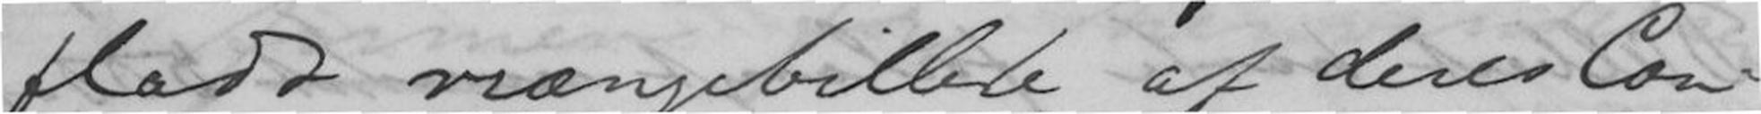fladt vrængebillede af Deres Con-
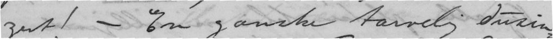zert! - En ganske tarvelig dusin
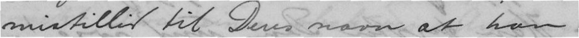mistillid til Deres navn at han
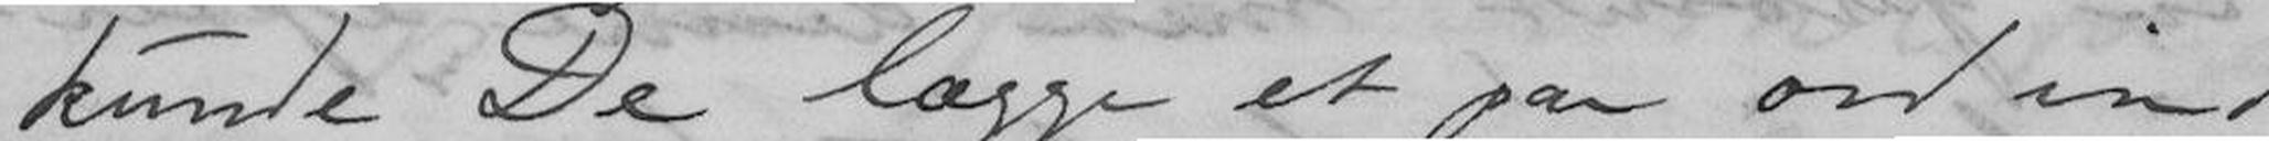kunde De lægge et par ord ind
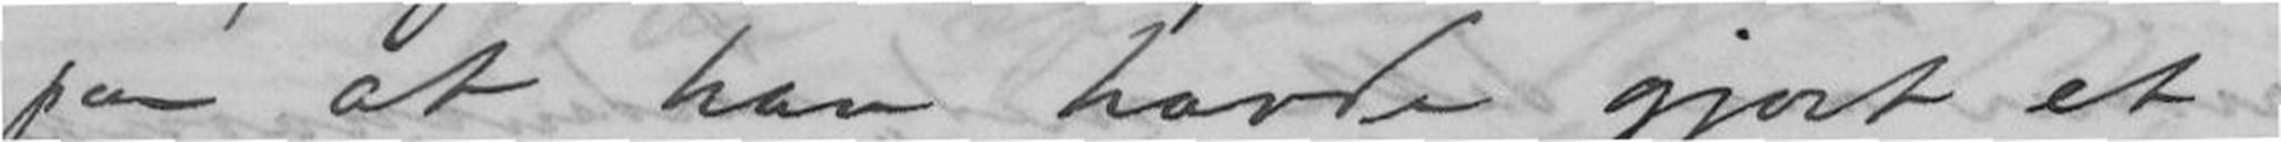paa at han havde gjort et
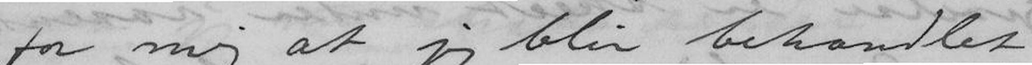for mig at jeg blir behandlet
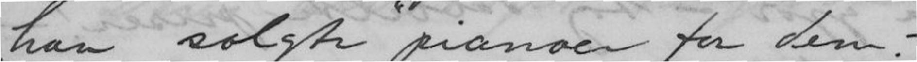han "solgte" pianoer for dem.
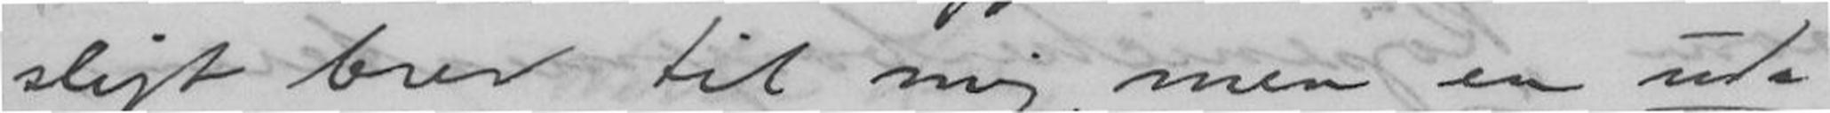sligt brev til mig, men en ud-
